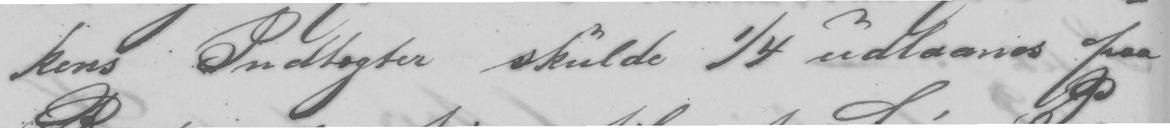kens Indtægter skulde ¼ udlaanes paa
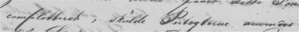completteret, skulde Integterne anvendes
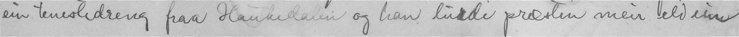ein tenestedreng fraa Hankedalen og han lurde præsten meir eld ein
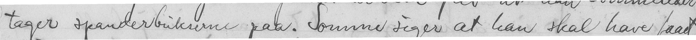tager spanderbukserne paa. Somme siger at han skal have faaet
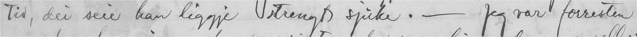tid, dei seie han liggje strengt sjuke. - Jeg var forresten
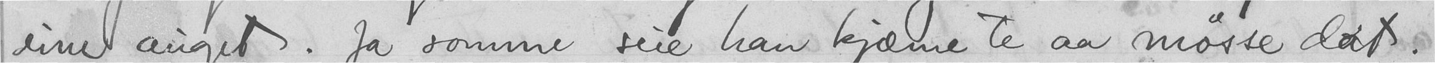eine auget. Ja somme seie han kjæme te aa møsse dat.
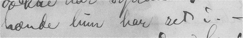hænde hun har ret i. -
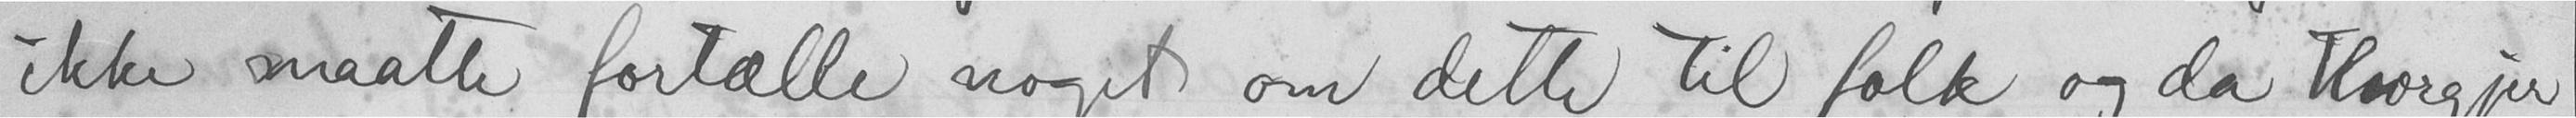ikke maate fortælle noget om dette til folk og da Thorgjer
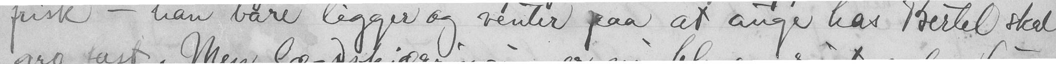frisk - han bare ligger og venter paa at auge has Bertel skal
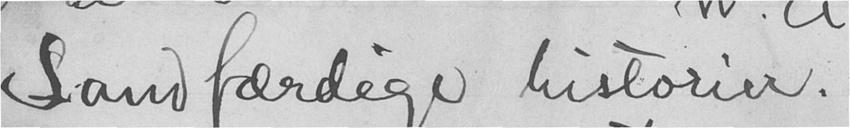Sandfærdige historier.
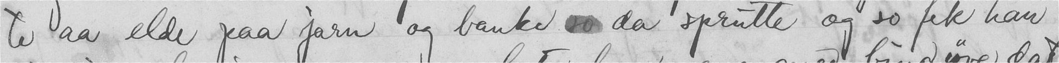te aa elde paa jarn og banke so da sprutte og so fek han
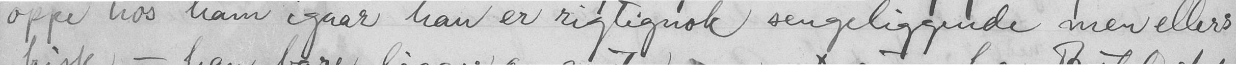oppe hos ham igaar han er rigtignok sengeliggende men ellers
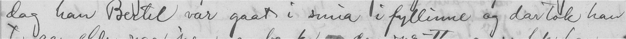dag han Bertel var gaat i smia i fyllinne og dar tok han
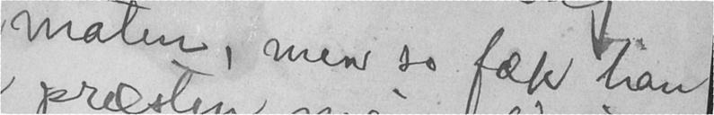maten, - men so fæk han
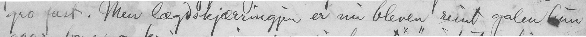gro fast. Men lægdskjærringjen er nu bleven reint galen hun
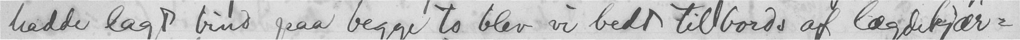hadde lagt bind paa begge to blev vi bedt tilbords af lægdekjær-
